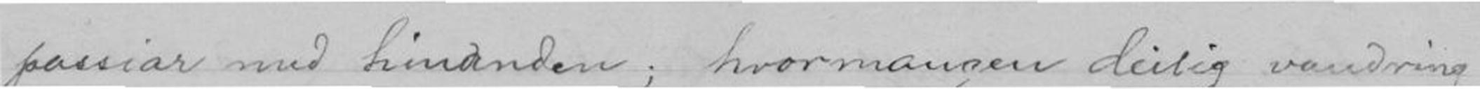passiar med hindanden; hvormangen deilig vandring
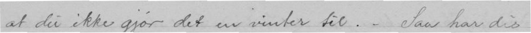at du ikke gjør det en vinter til. - Saa har du
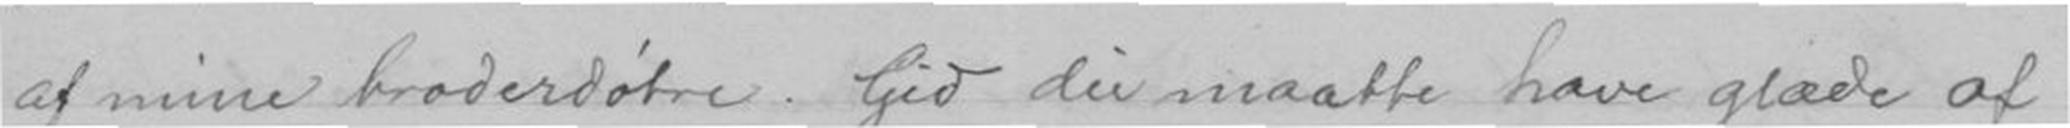af mine broderdøtre. Gid du maatte have glæde af
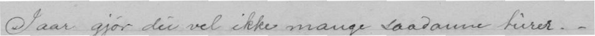- Iaar gjør du vel ikke mange saadanne turer. -
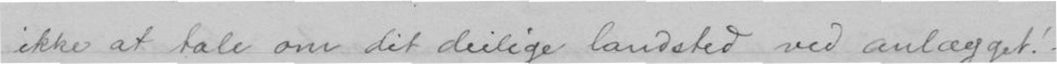ikke at tale om dit deilige landsted ved anlægget!
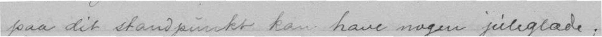paa dit standpunkt kan have nogen juleglæde;
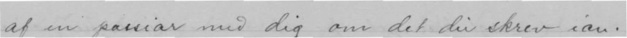af en passiar med dig om det du skrev ian-
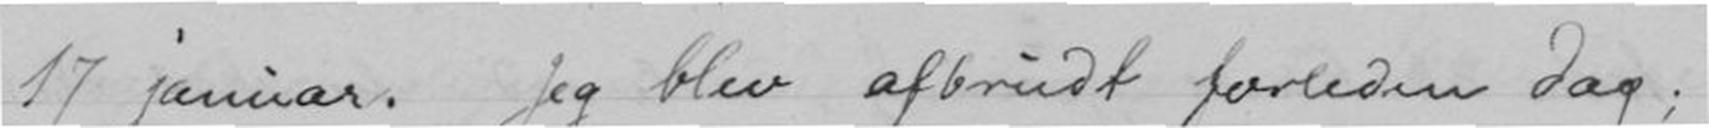17 januar. Jeg blev afbrudt forleden dag;
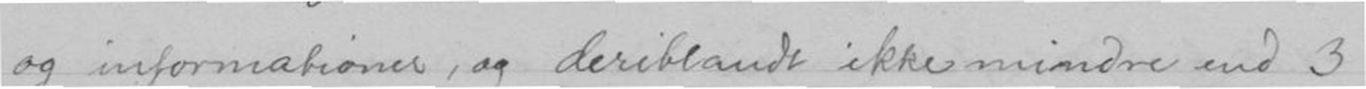og informationer. og deriblandt ikke mindre end 3
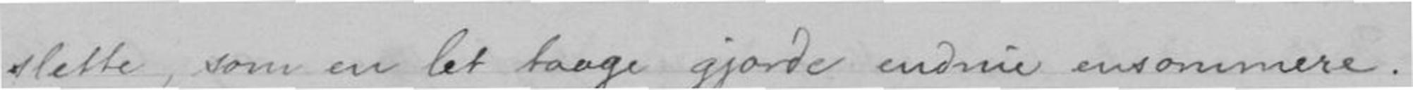slette, som en let taage gjorde endnu ensommere.
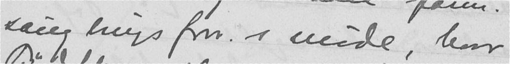saugbrugsforn.s møde, hvor
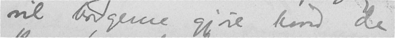vil borgerne gjøre hvad de
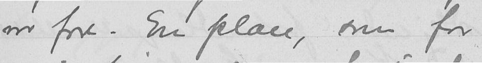var fore. En plan, som for
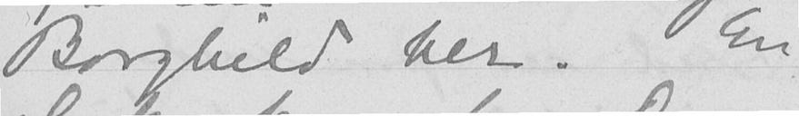Borghild her. En
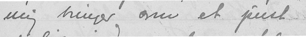mig bringer som et pust
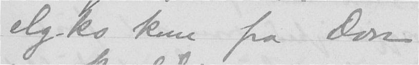elg-ko kom fra Don
